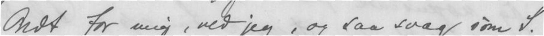Ondt for mig, ved jeg, og saa svag som S.
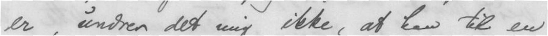er, undren det mig ikke, at han til en
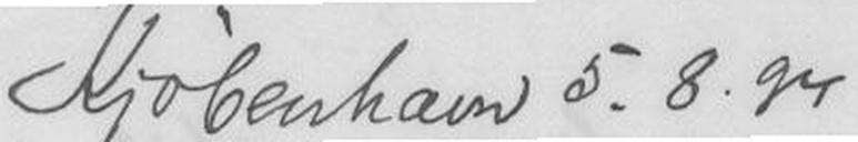Kjøbenhavn 5. 8. 94
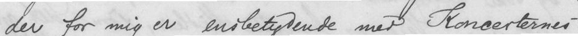der for mig er ensbetydende med Koncerternes
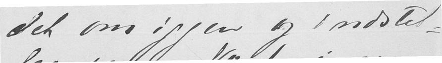det om igjen og indstil-
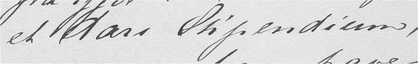et Aars Stipendium,
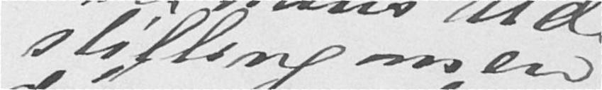stilling men
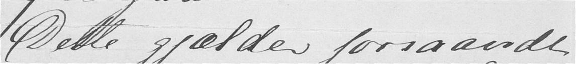Dette gjælder forsaavidt
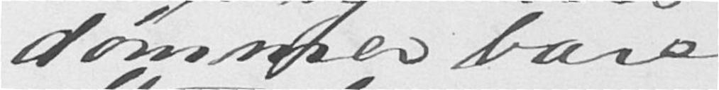dømmer bare
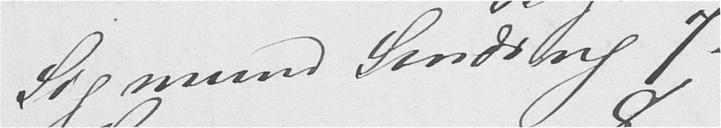Sigmund Sinding 7.
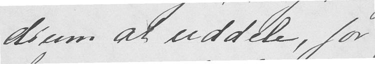dium at uddele, for jeg
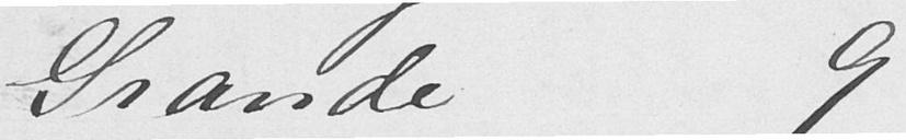Grande 9
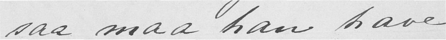saa maa han have
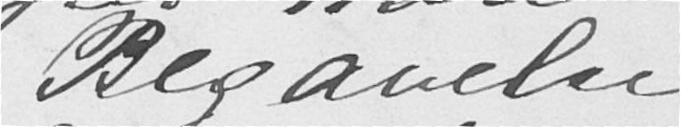Begavelse.
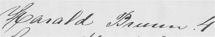Harald Bruun 4.
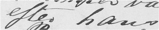efter hans
